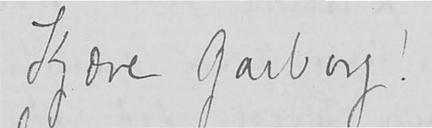Kjære Garborg!
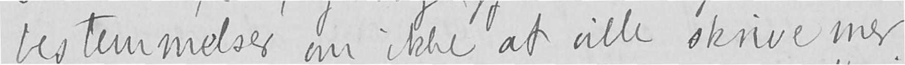bestemmelser om ikke at ville skrive mer.
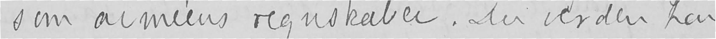som arméens regnskaber. Du verden har
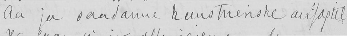Aa ja saadanne kunstneriske anfægtel-
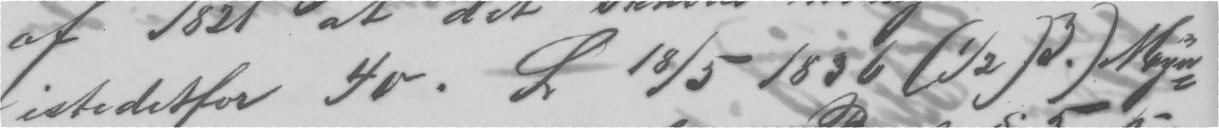istedetfor 40. L 18/5 1836 (½ ẞ.) Myn-
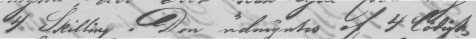4 Skilling. Den udmyntes af 4 løligt
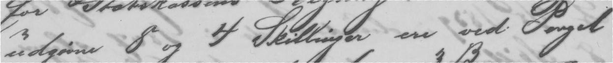udgivne 8 og 4 Skillinger en ved Pengel
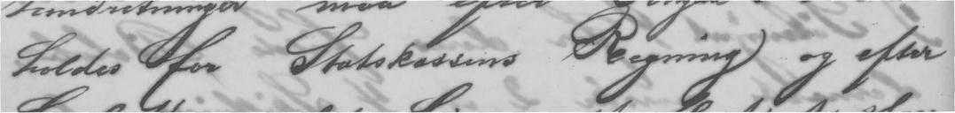holdes for Statskassens Regning og efter
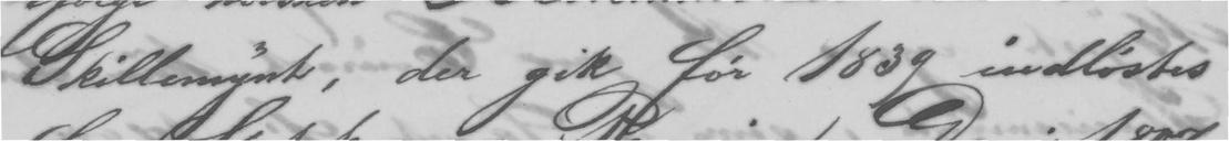Skillemynt, der gik før 1839: indløstes
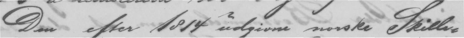Den efter 1814 udgivne norske Skille-
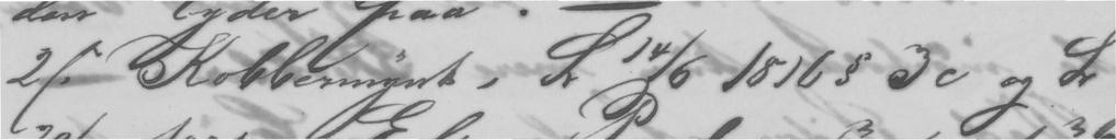21 Kobbermynt, h 14/6 1816 §3e og L
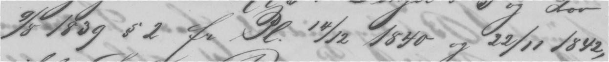9/8 1839 §2 fr Pl. 14/12 1840 og 22/11 1842,
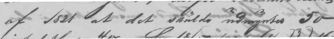af 1821 at det skulde udmyntes 50
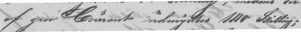af grov Courant udmyntes 1110 Skilling;
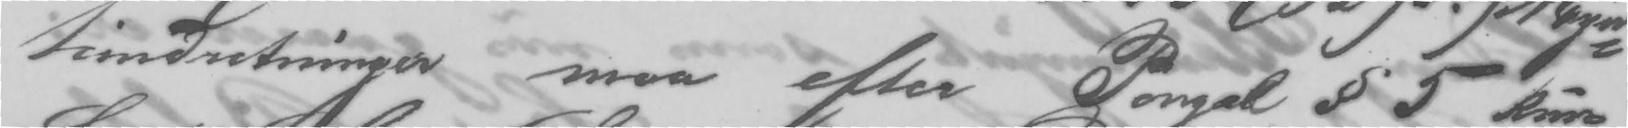tindretninger maa efter Pengel §5 kun
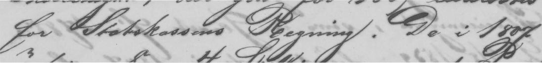for Statskassens Regning. De i 1807
